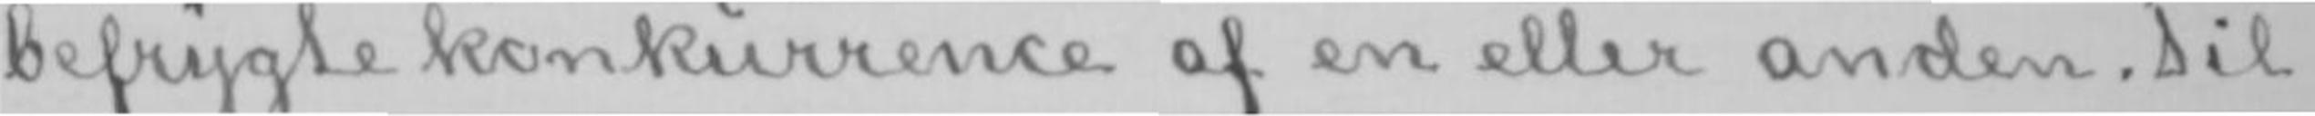befrygte konkurrence af en eller anden. Til
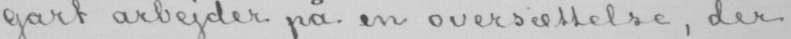gart arbejder på en oversættelse, der
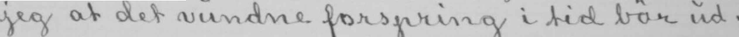jeg at det vundne forspring i tid bør ud-
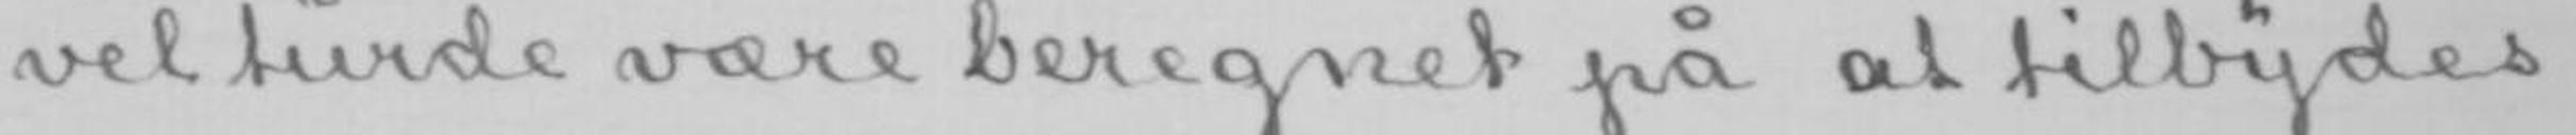vel turde være beregnet på at tilbydes
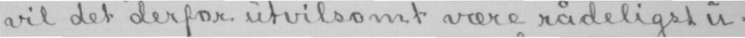vil det derfor utvivlsomt være rådeligst u-
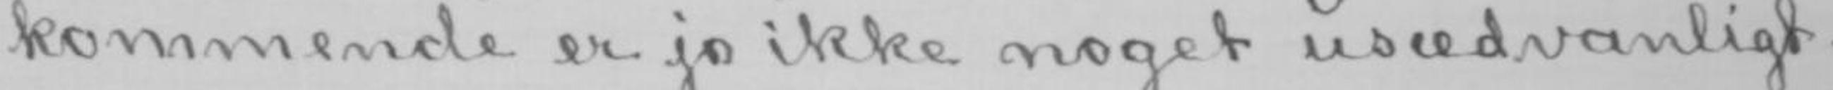kommende er jo ikke noget usædvanligt.
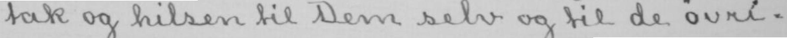tak og hilsen til Dem selv og til de øvri-
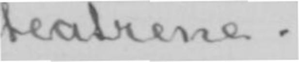teatrene.
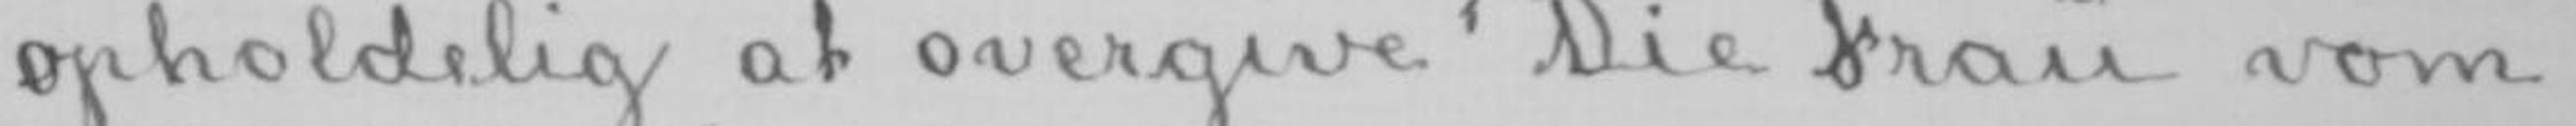opholdelig at overgive «Die Frau vom
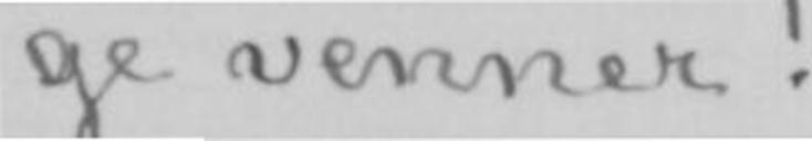ge venner!
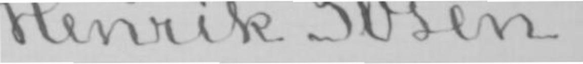Henrik Ibsen.
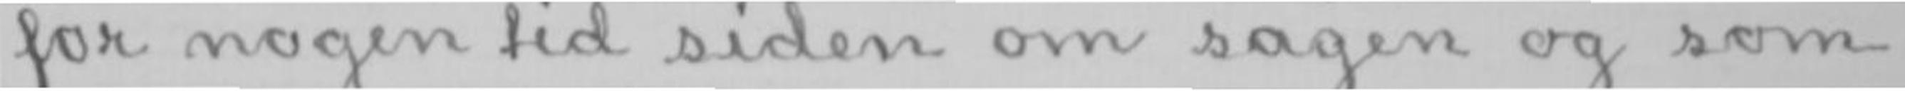for nogen tid siden om sagen og som
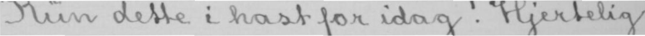Kun dette i hast for i dag! Hjertelig
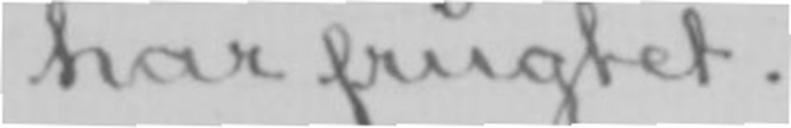har frugtet.
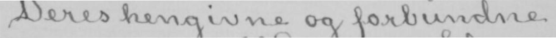Deres hengivne og forbundne
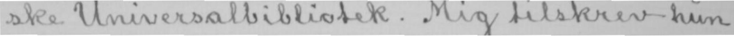ske Universalbibliotek. Mig tilskrev hun
